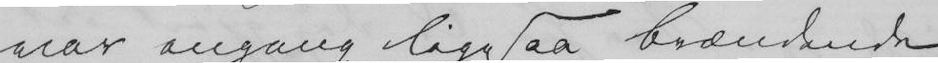var engang ligesaa brændende
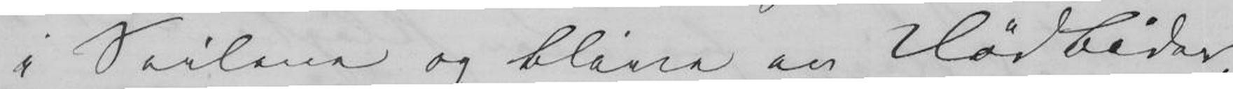i seilerne og blive en Dødbider,
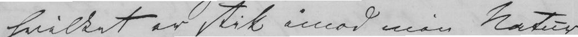hvilket er stik imod min Natur
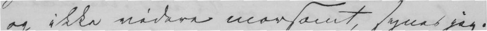og ikke videre morsomt, synes jeg.
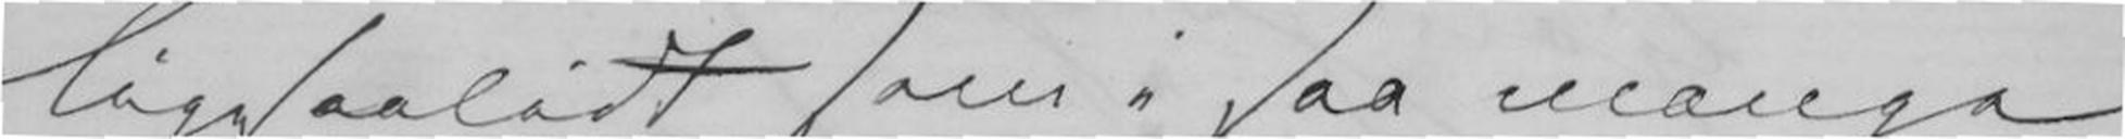ligesaalidt som i saa mange
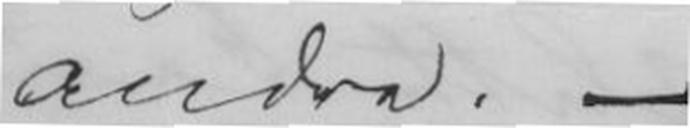andre. -
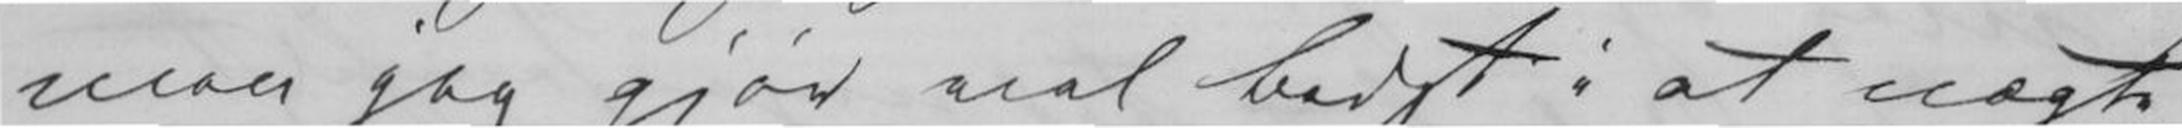naar jeg gjør vel bedst i at nægte
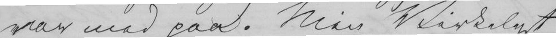var med paa. Min Virkelyst
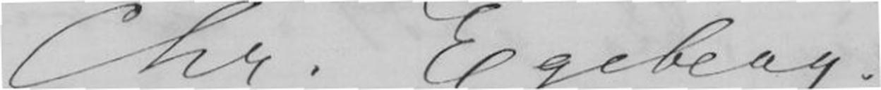Chr. Egeberg
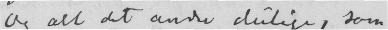Og alt det andre deilige, som
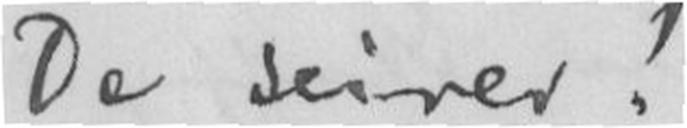De seirer!
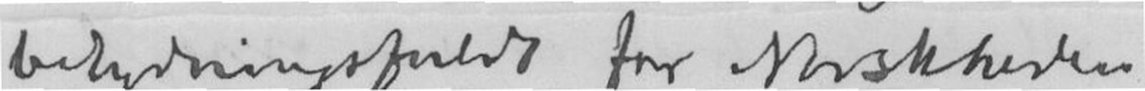betydningsfuldt for Norskheden
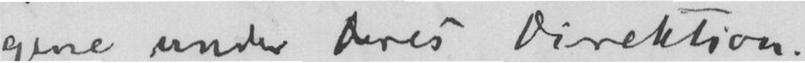gene under Deres Direktion.
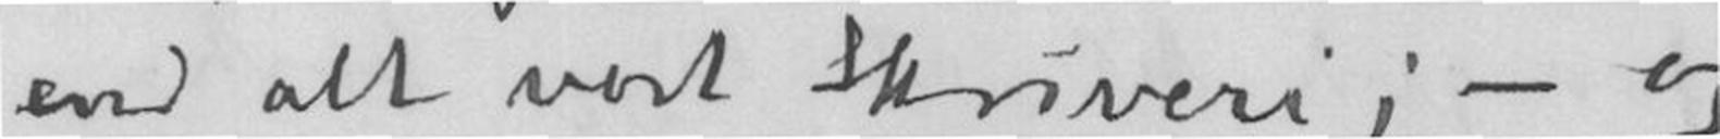end alt vort Skriveri; - og
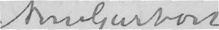Arne Garborg
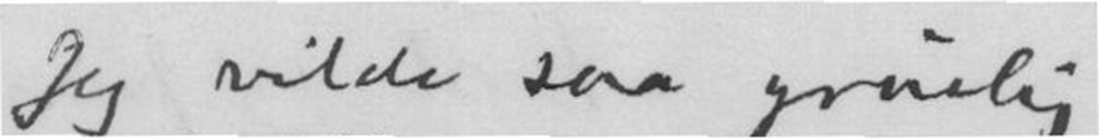Jeg vilde saa gruelig
